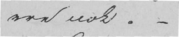ere nok. -
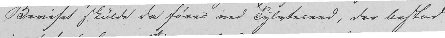Beviset skulde da føres ved Tylvteseed, der bestod
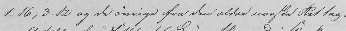1-16, 3-12 og de øvrige fra den ældre norske Ret tag-
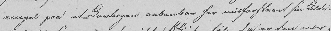empel paa at Lovbogen aabenbar har misforstaaet sin Kilde.
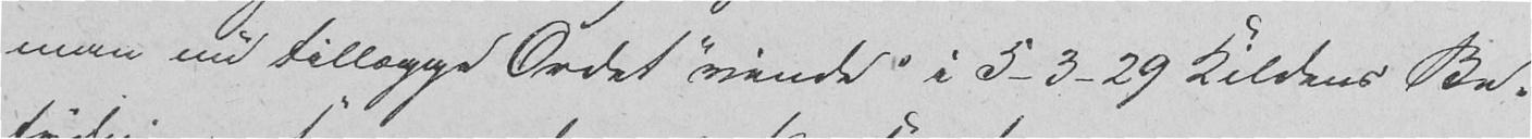man nu tillægge Ordet "vinde" i 5-3-29 Kildens Be-
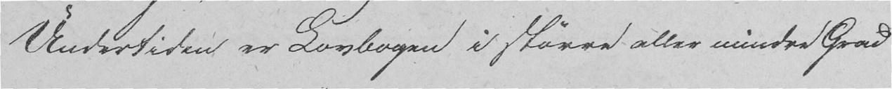Undertiden er Lovbogen i større aller mindre Grad
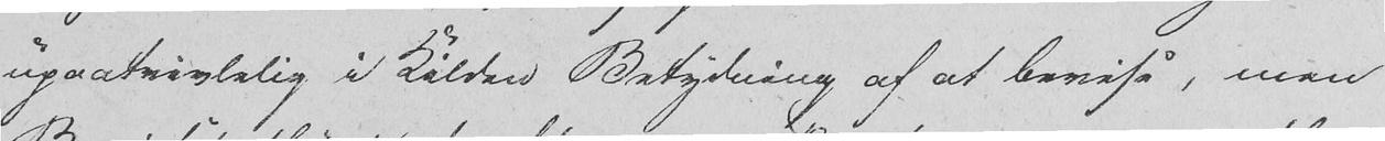upaatvivlelig i Kilden Betydning af at bevise, men
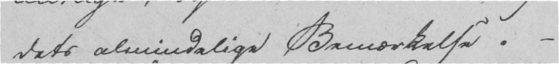dets almindelige Bemærkelse. -
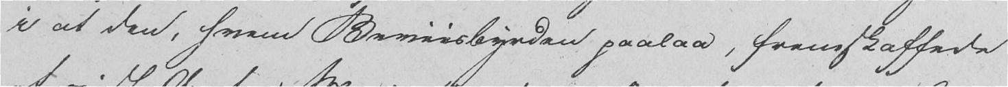i at den, hvem Beviisbyrden paalaa, fremskaffede
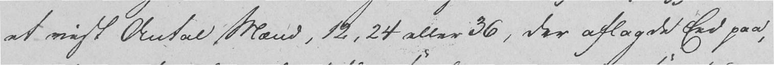et vist Antal Mænd, 12, 24 eller 36, der aflagde Eed paa,
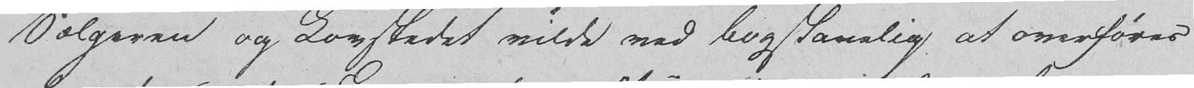Sælgeren og Lovstedet vilde ved bogstavelig at overføres
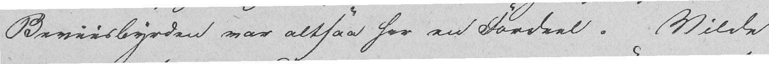Beviisbyrden var altsaa her en Fordeel. Vilde
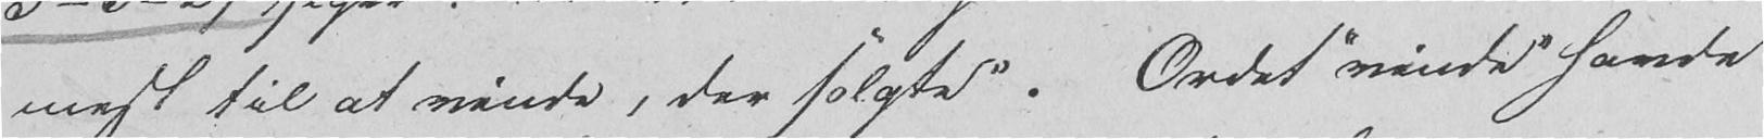mest til at vinde, der solgte". Ordet "vinde" havde
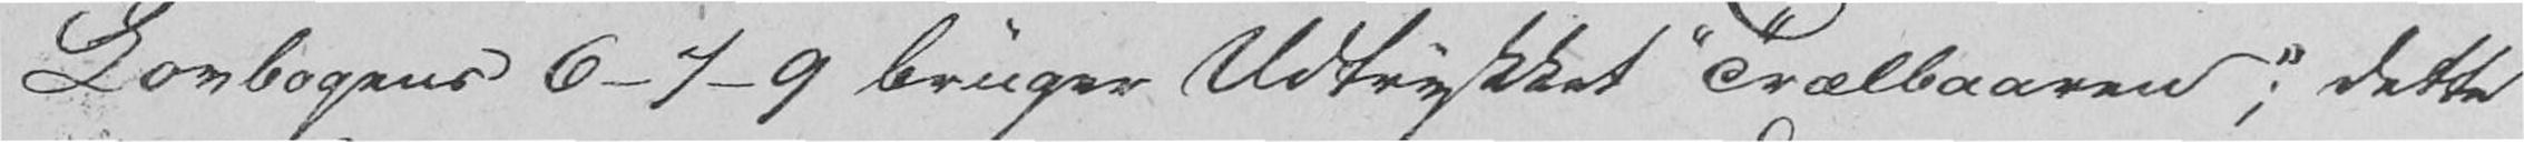Lovbogens 6-7-9 bruger Udtrykket "Trælbaaren"; dette
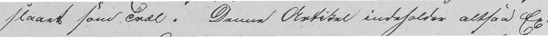slaaet som Træl. Denne Artikel indeholder altsaa Ex-
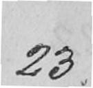23.
